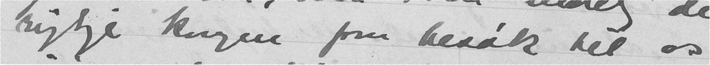rigtige kongen paa besøk til os
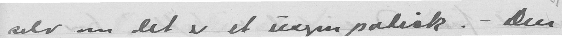selv om det er et uegenpatisk. - Den
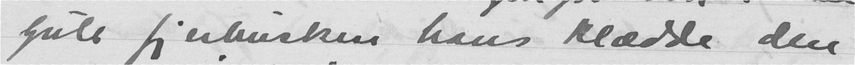gule fjerhusken hans klædde den
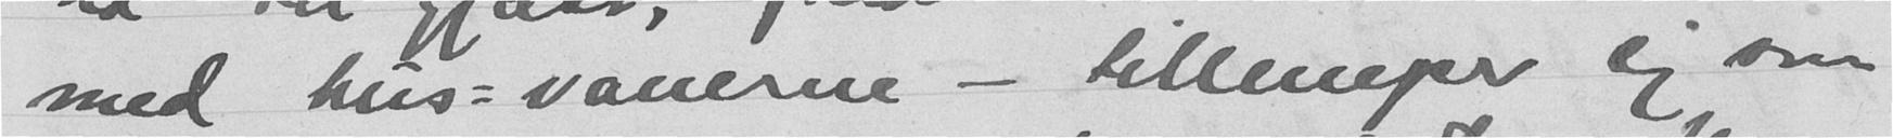med hus-vanerne - tillemper sig som
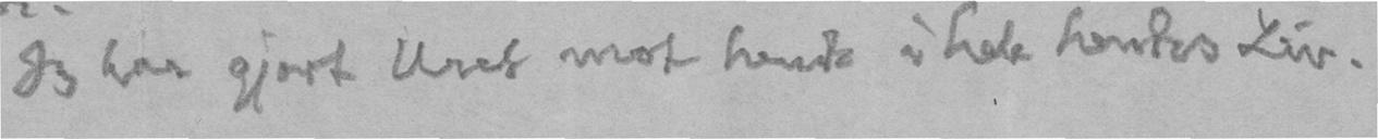Jeg har gjort Uret mot hende i hele hendes Liv.
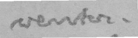venter.
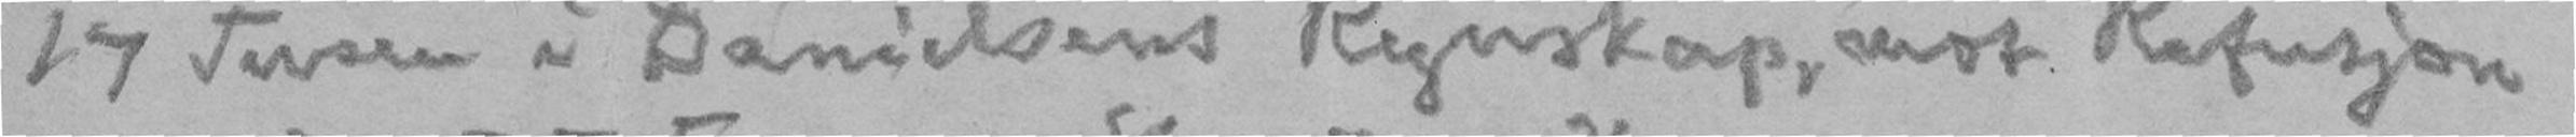17 Tusen i Danielsens Regnskap, mot Refusjon
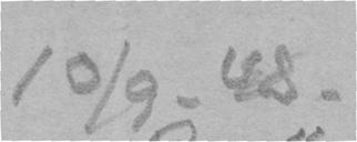10/9. 48.
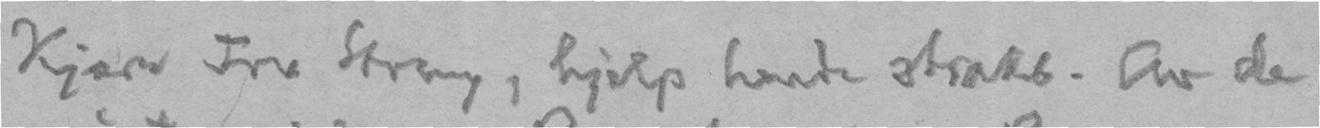Kjære Fru Stray, hjelp hende straks. Av de
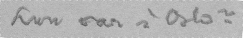hun var i Oslo?
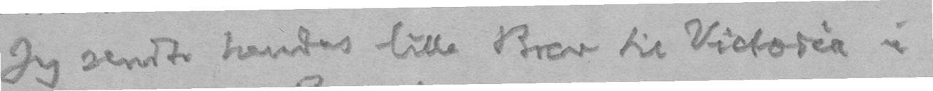Jeg sendte hendes lille Brev til Victoria i
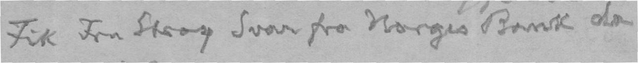Fik Fru Stray Svar fra Norges Bank da
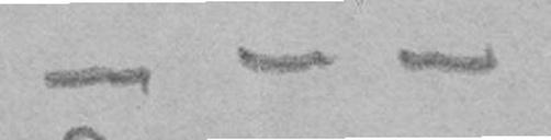- - -
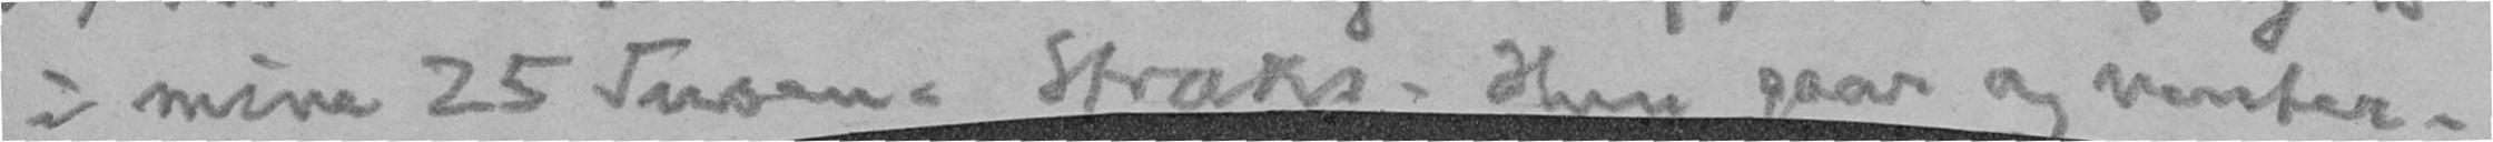i mine 25 Tusen. Straks. Hun gaar og venter.
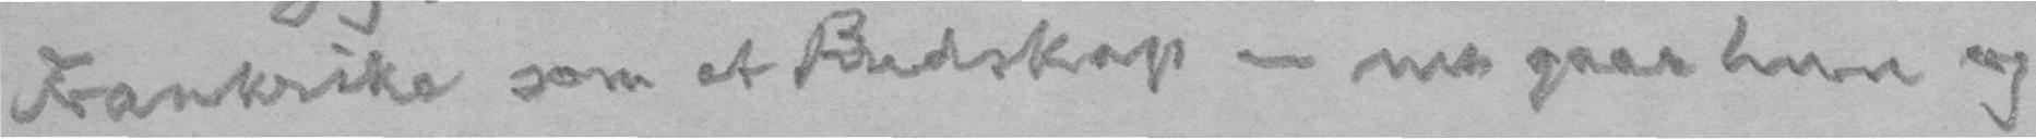Frankrike som et Budskap - nu gaar hun og
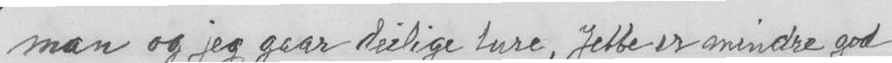man og jeg gaar deilige turer, Jette er mindre god
